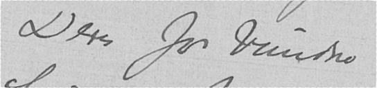Deres forbundne
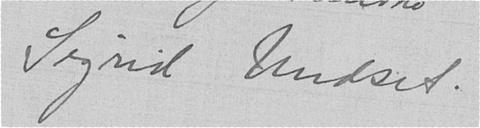Sigrid Undset.
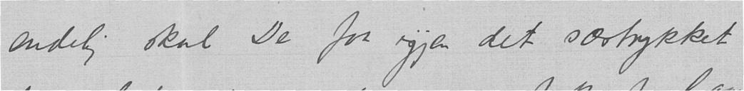endelig skal De faa igjen det særtrykket
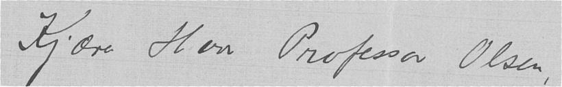Kjære Herr Professor Olsen,
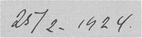25/2-1924.
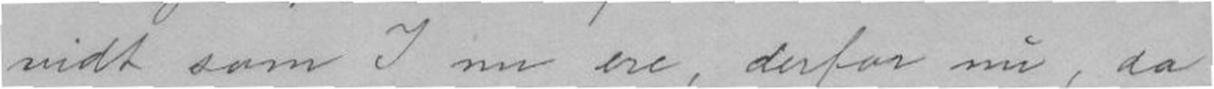vidt som I nu ere, derfor nu, da
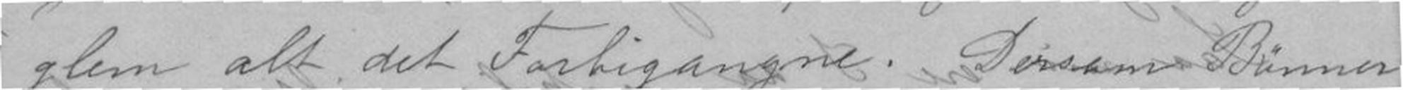glem alt det Forbigangne. Dersom Bønner
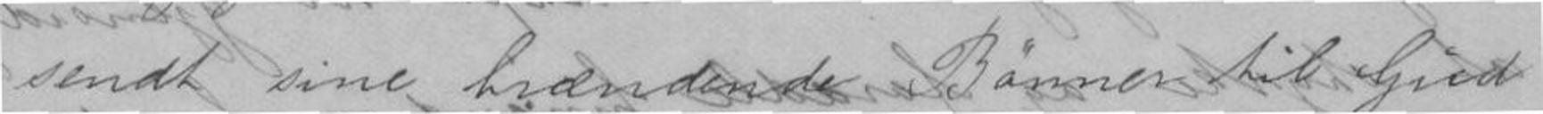sendt sine brændende Bønner til Gud
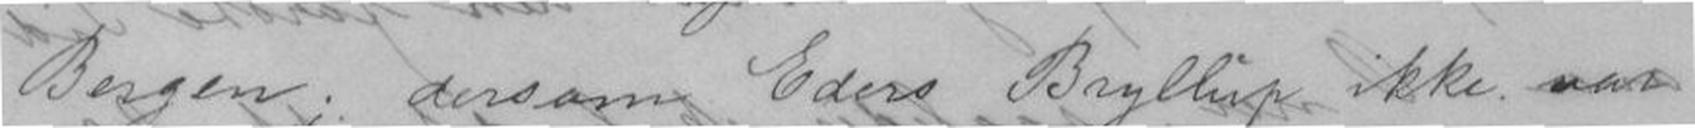Bergen; dersom Eders Bryllup ikke var
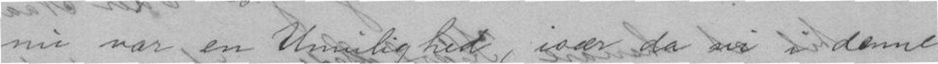nu var en Umulighed, især da vi i denne
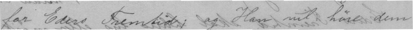for Eders Fremtid; og Han vil høre dem
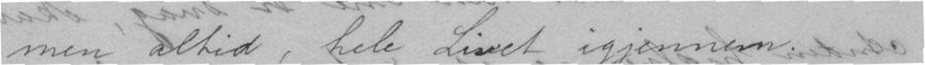men altid, hele livet igjennem.
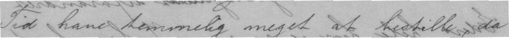Tid have temmelig meget at bestille, da
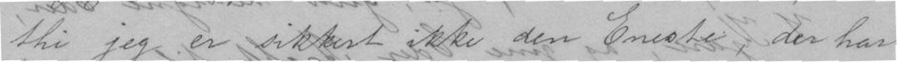thi jeg er sikkert ikke den Eneste; der har
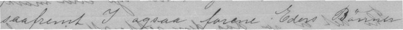saafremt I ogsaa forene Eders Bønner
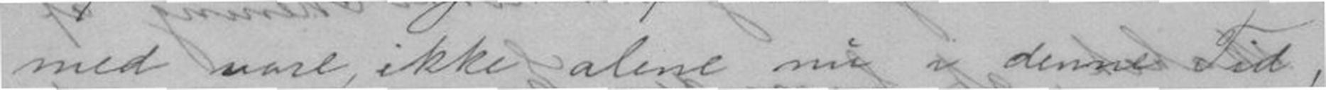med vore, ikke alene nu i denne Tid,
